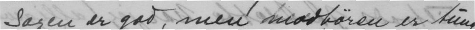Sagen er god, men modbøren er tund,
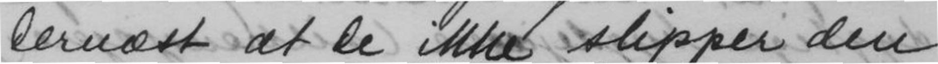Dernæst at De ikke slipper den
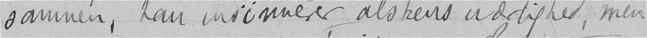sammen, han insinuerer alskens uærlighed, men
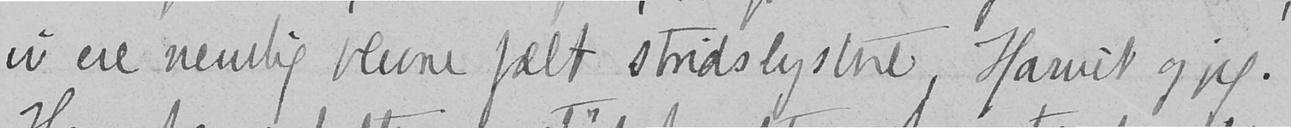vi ere nemlig blevne fælt stridslystne, Harriet og jeg.
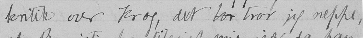kritik over Krog, det bor tror jeg neppe,
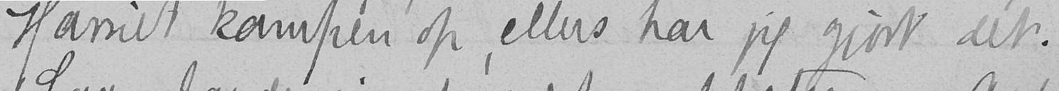Harriet kampen op, ellers har jeg gjort det.
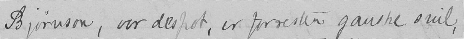Bjørnson, vor despot, er forresten ganske snil,
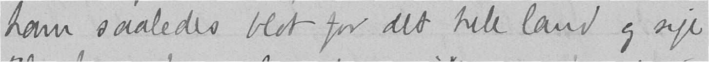ham saaledes blot for det hele land og sige
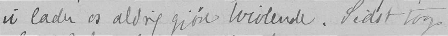vi lader os aldrig gjøre tvivlende. Sidst tog
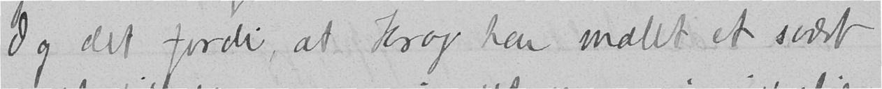Og det fordi, at Krog har malet et svært
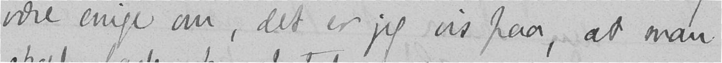være enige om, det er jeg vis paa, at man
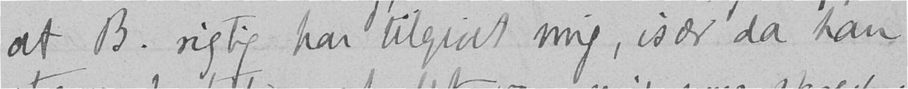at B. rigtig har tilgivet mig, især da han
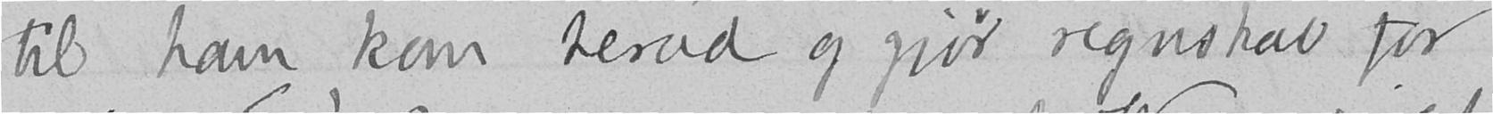til ham, kom herud og gjør regnskab for
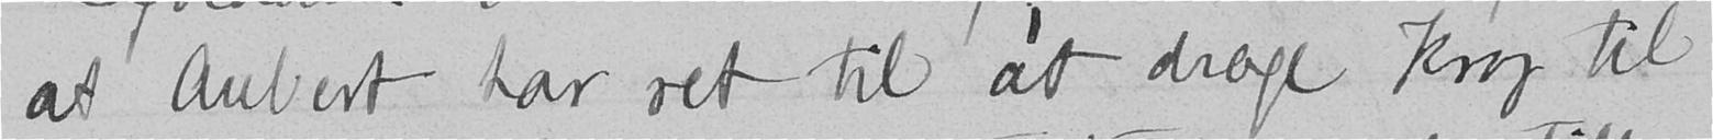at Aubert har ret til at drage Krog til
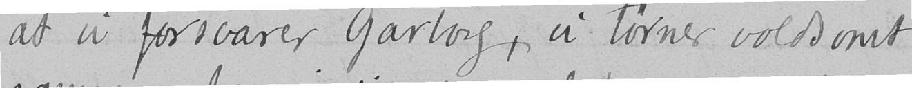at vi forsvarer Garborg, vi tørner voldsomt
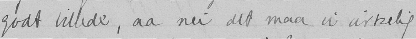godt billede, aa nei det maa vi virkelig
